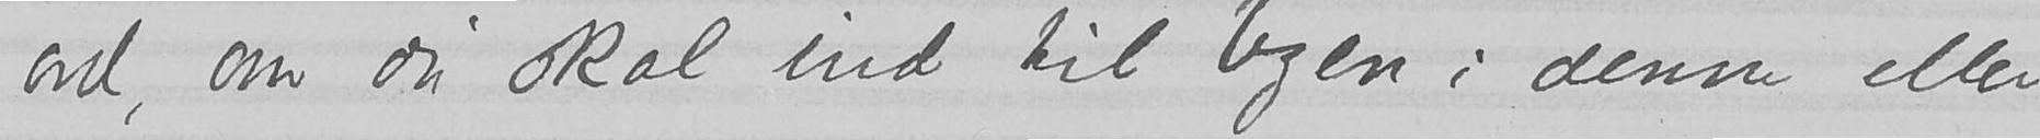ord, om du skal ind til byen i denne eller
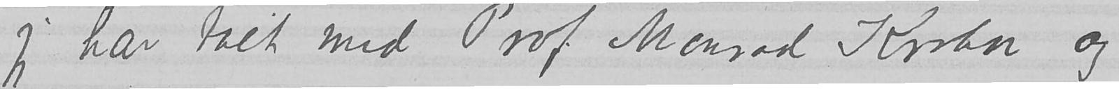jeg har talt med Prof. Monrad Krohn og
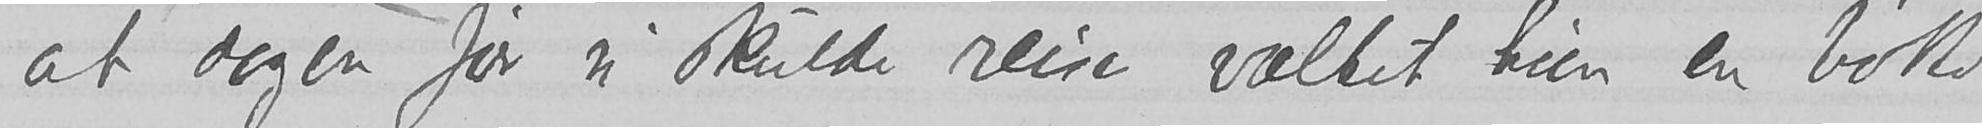at dagen før vi skulde reise væltet hun en bøtte
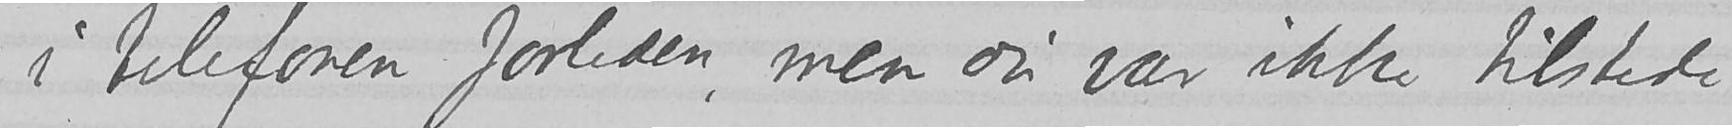i telefonen forleden, men du var ikke tilstede
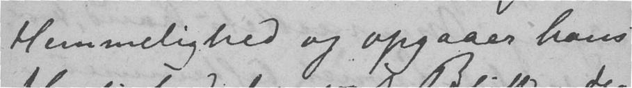Hemmelighed og opgaaer hans
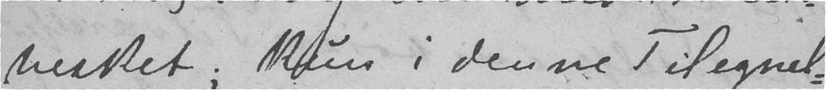nesket. Kun i denne Tilegne-
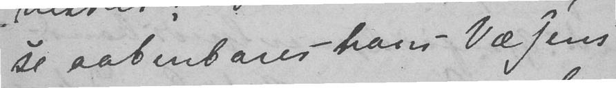se aabenbares hans Væsens
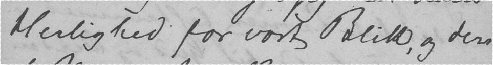Herlighed for vort Blik, og deri
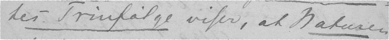tes Trinølge viser, at Naturen
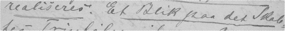realiseres. Et Blik paa det Skab-
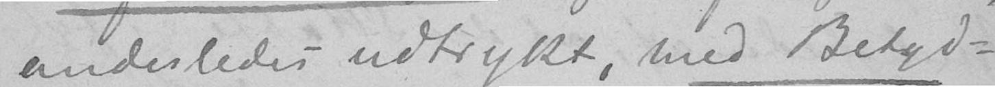anderledes udtrykt, med Betyd-
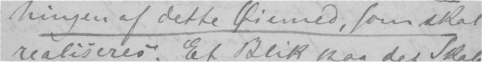ningen af dette Øiemed, som skal
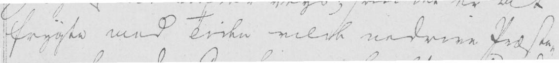frygte med Tiden vilde nedrive Præste-
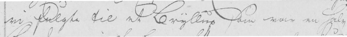vi fulgte til et Bryllup som var en halv
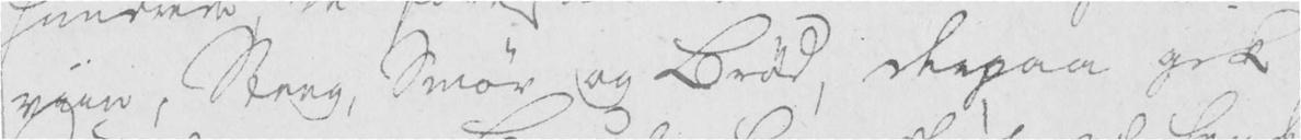viin, Steeg, Smør og Brød, derpaa gik
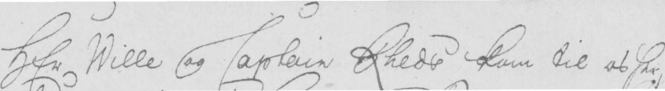Hr Wille og Captain Rheds kom til os her,
